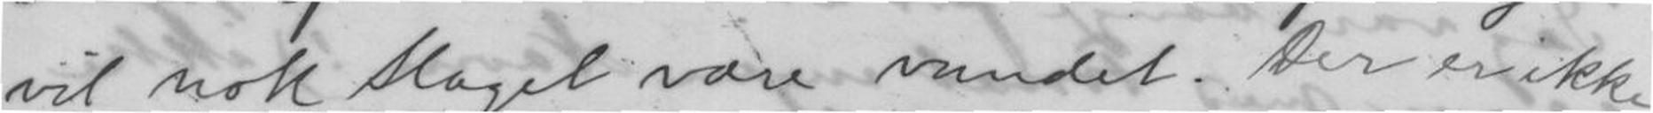vil nok Slaget være vundet. Der er ikke
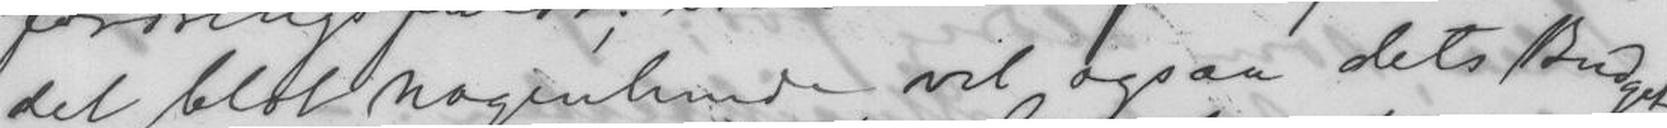det blot nogenlunde vil ogsaa dets Budget
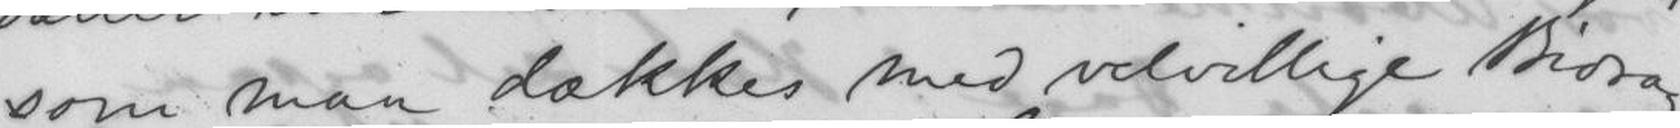som maa dækkes med velvillige Bidrag
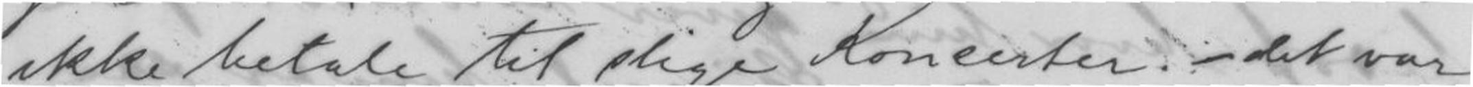ikke betale til slige Koncerter. - det var
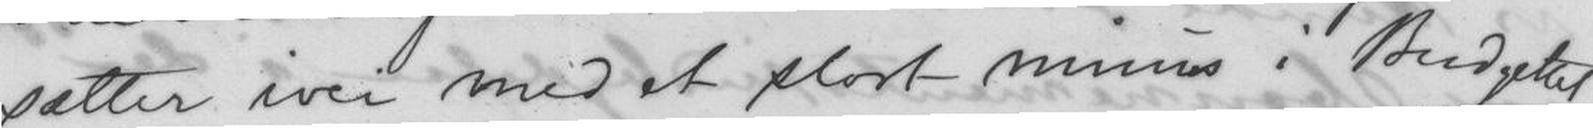sætter ivei med et stort minus i Budgettet
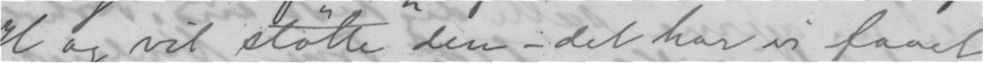H og vil støtte den - det har vi faaet
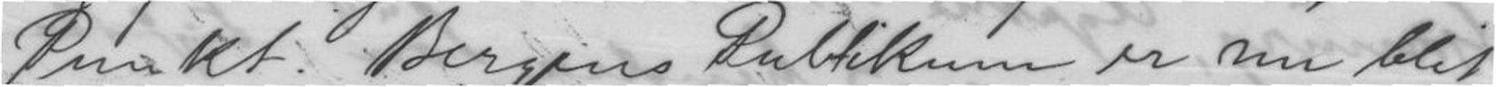Punkt. Bergens Publikum er nu blit
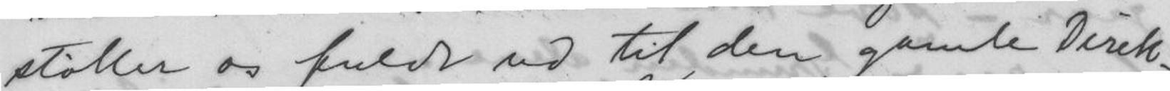støtter os fuldt ut til den gamle Direk-
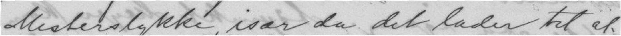Mesterstykke, især da det lader til at
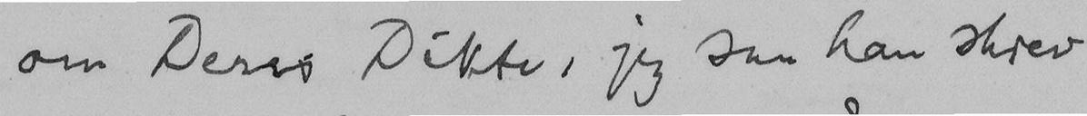om Deres Dikte, jeg saa han skrev
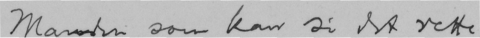Manden som kan si det rette
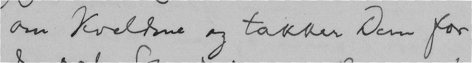om Kveldene og takker Dem for
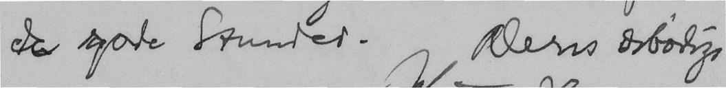de gode Stunder. Deres ærbødige
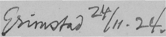Grimstad 24/11. 24.
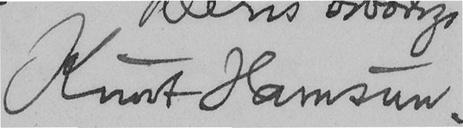Knut Hamsun.
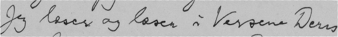Jeg læser og læser i Versene Deres
